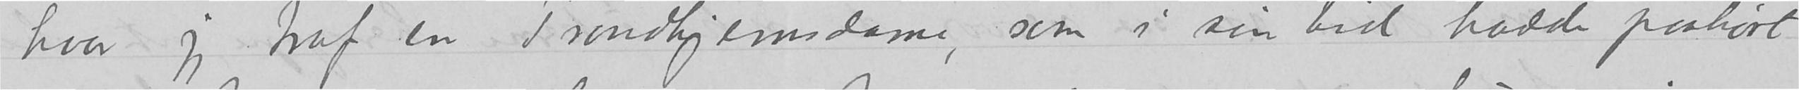hvor jeg traf en Trondhjemsdame, som i sin tid hadde paahørt
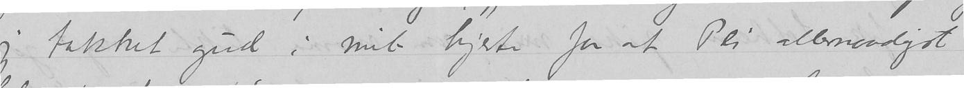- jeg takket gud i mit hjerte for at Pei allernondigst
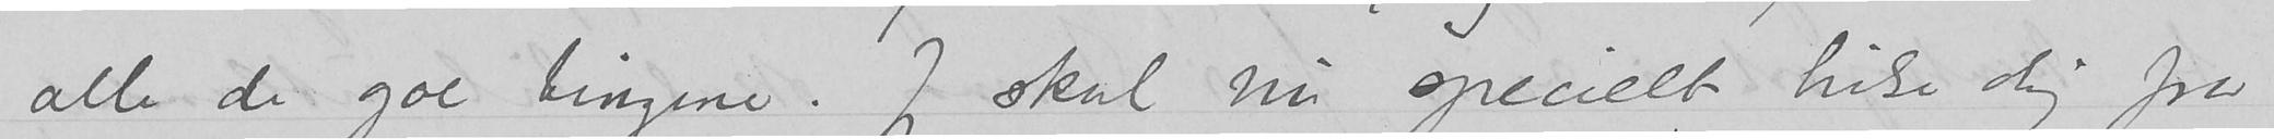alle de goe tingene. Jeg skal nu specielt hilse dig fra
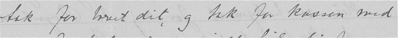tak for brevet dit og tak for kassen med
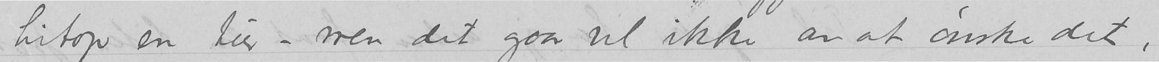hitop en tur – men det gaar vel ikke an at ønske det,
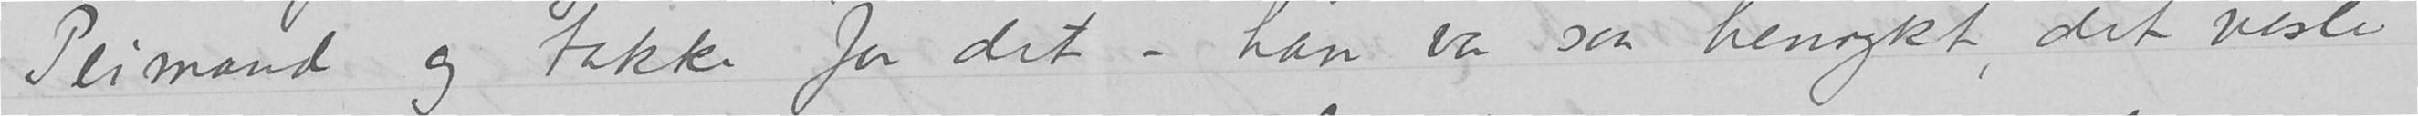Peimand og takke for det – han var saa henrykt, det vesle
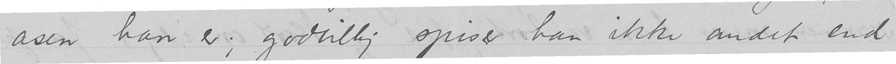asen han er; godbillig spiser han ikke andet end
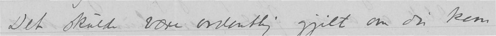Det skulde være ordentlig gjilt om du kom
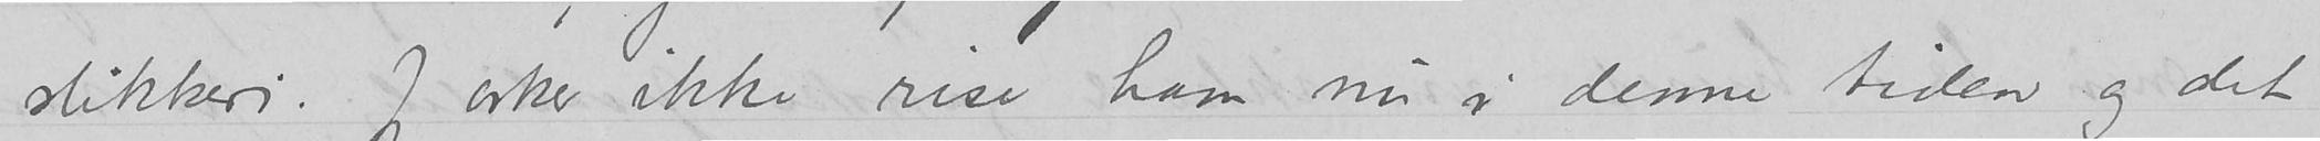slikkeri. Jeg orker ikke vise ham nu i denne tiden og det
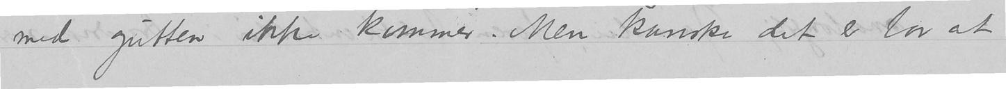med gutten ikke kommer. Men kanske det er lov at
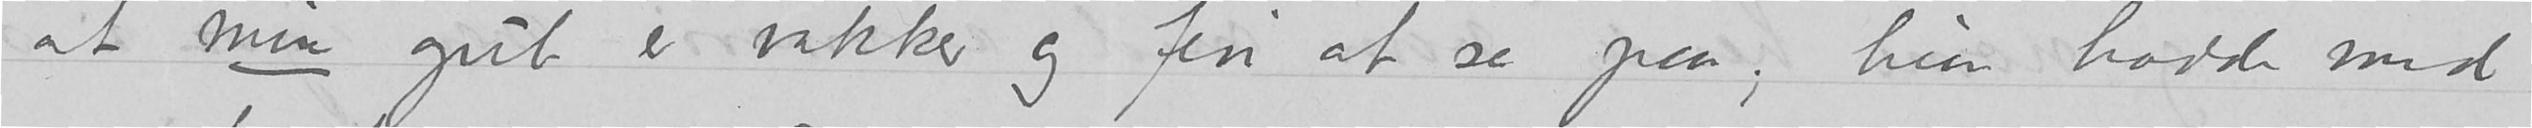at min gut er vakker og fin at se paa; hun hadde med
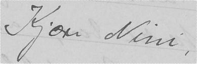Kjære Nini,
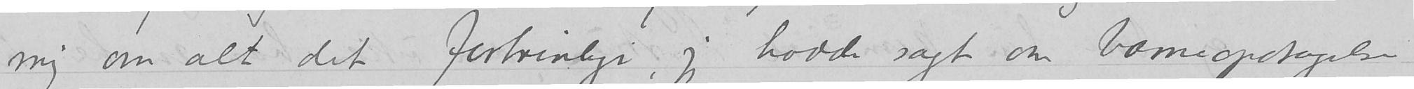mig om alt det fortrinlige jeg hadde sagt om barneopdragelse
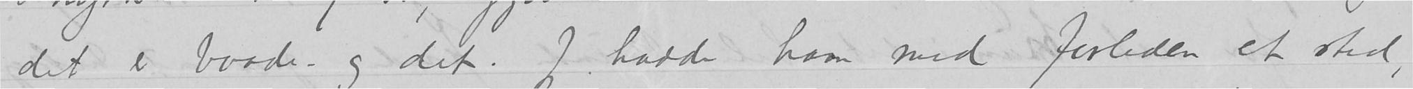det er baade og det. Jeg hadde ham med forleden et sted,
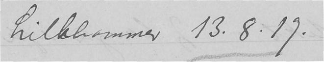Lillehammer 13.8.19.
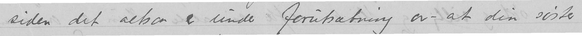siden det altsaa er under forutsætning av at din søster
